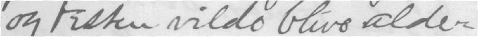og Festen vilde blive alde-
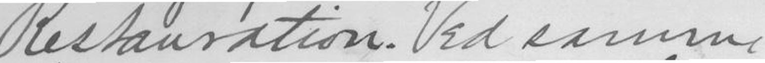Restauration. Ved samme
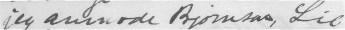jeg anmode Bjørnson, Lie
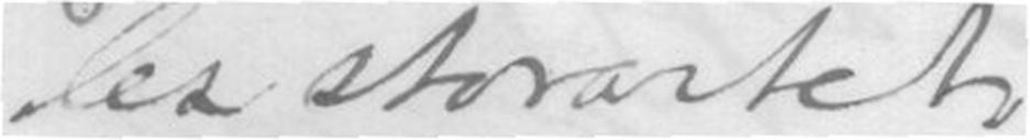es storartet.
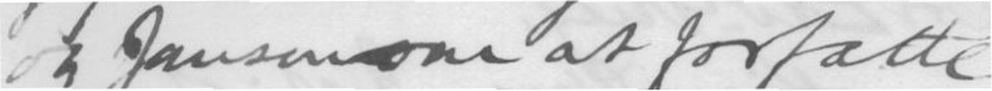og Janson om at forfatte
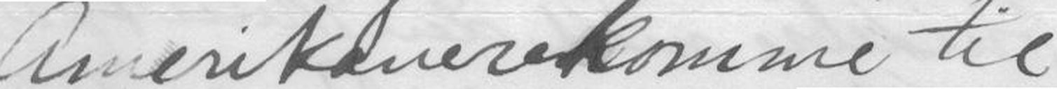Amerikanere komme til
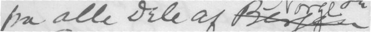fra alle Dele af Bergen Norge
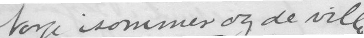Norge isommer og de ville
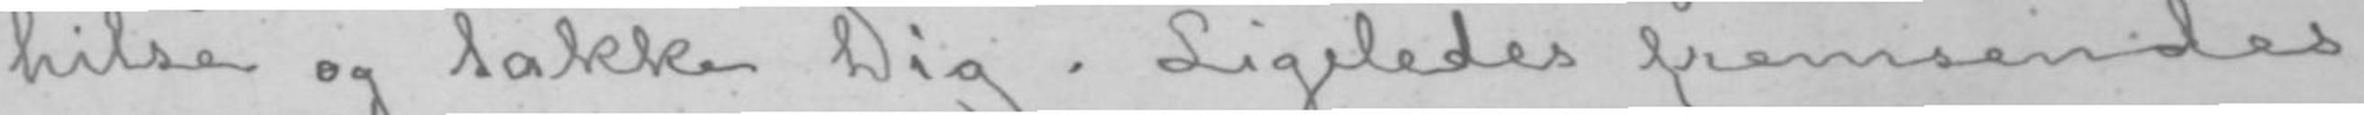hilse og takke Dig. Ligeledes fremsendes
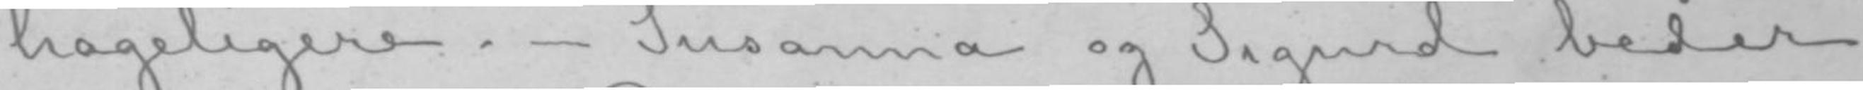hageligere. - Susanna og Sigurd beder
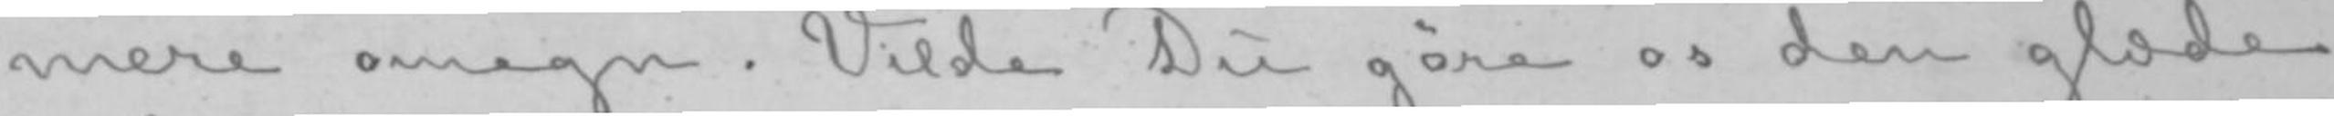mere omegn. Vilde Du gøre os den glæde
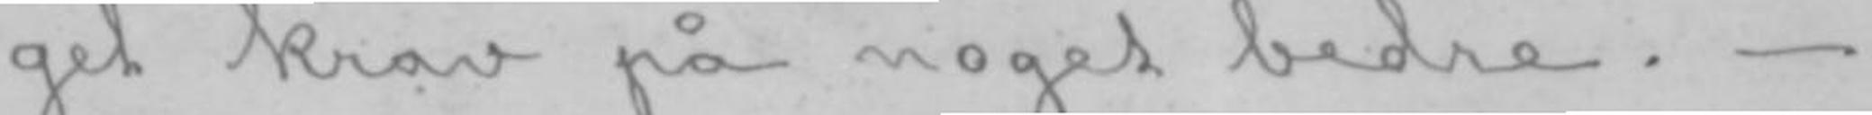get krav på noget bedre.-
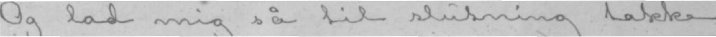Og lad mig så til slutning takke
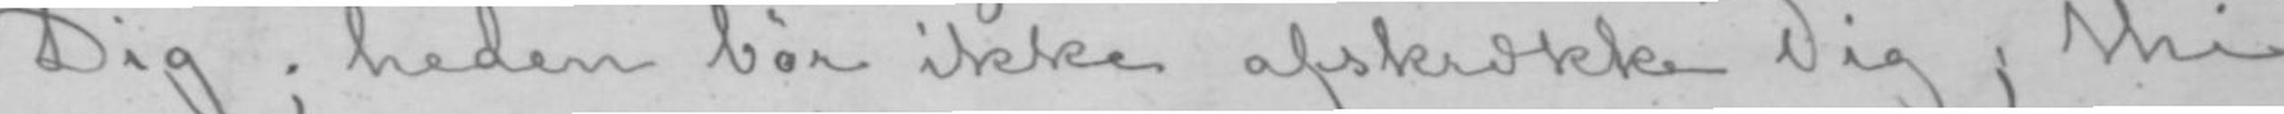Dig; heden bør ikke afskrække Dig; thi
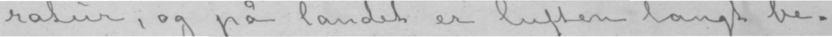ratur, og på landet er luften langt be-
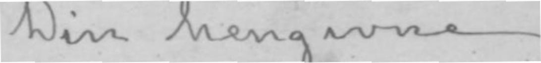Din hengivne
